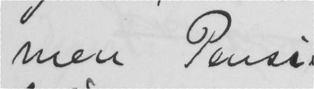men Pensi
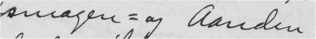smagen - og Aanden
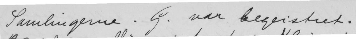Samlingerne. G. var begeistret.
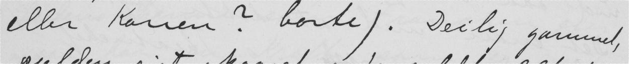eller Konen? borte). Deilig gammel,
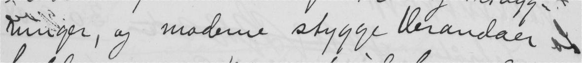ninger, og moderne stygge Verandaer
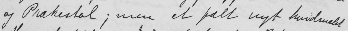og Prækestol; men et fælt nyt hvidmalet
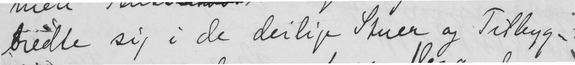tredte sig i de deilige Stuer og Tilbyg-
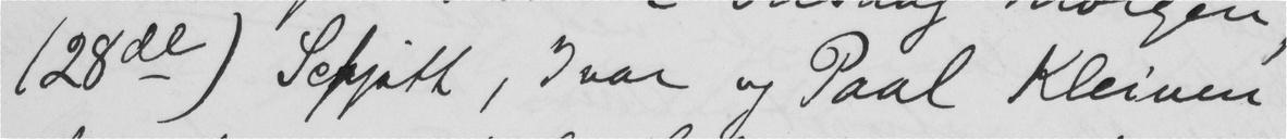(28de) Schjøtt, Ivar og Paal Kleiven
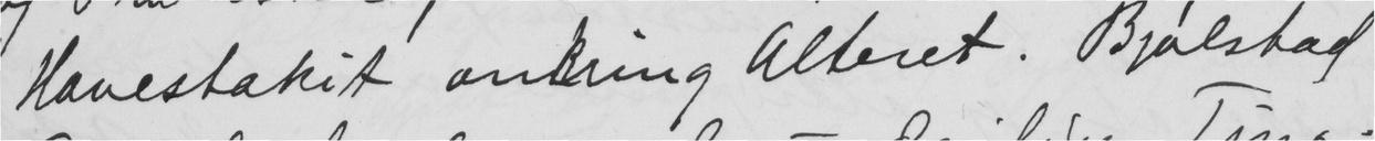Havestakit omring Alteret. Bjølstad
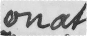onat
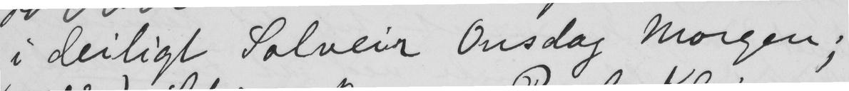i deiligt Solveir Onsdag Morgen;
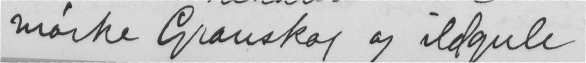mørke Granskog og ildgule somm
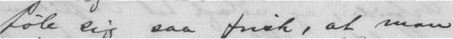føle sig saa frisk, at man
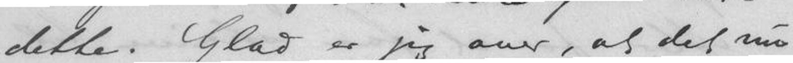dette. Glad er jeg over, at det nu
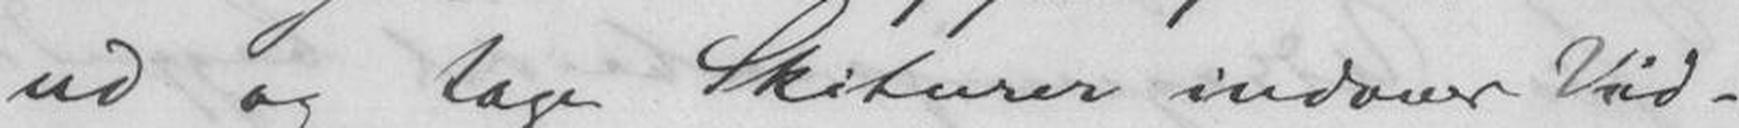ud og tage Skiturer indover Vid-
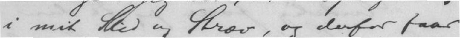i mit Slid og Stræv, og derfor faar
